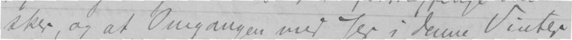sker, og at Omgangen med Jer i denne Vinter
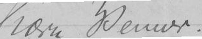Kære Venner.
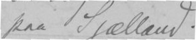paa Sjælland
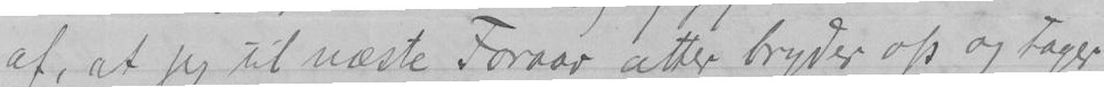af, at jeg til næste Foraar atter bryder op og tager
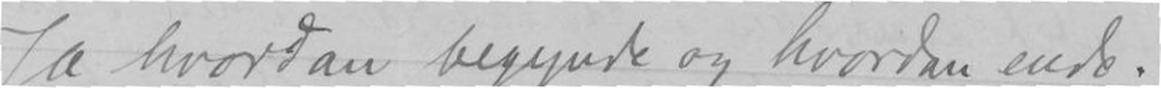Ja hvordan begynde og hvordan ende.
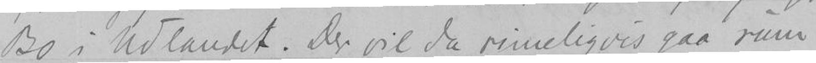Bo i Udlandet. Der vil da rimeligvis gaa rum
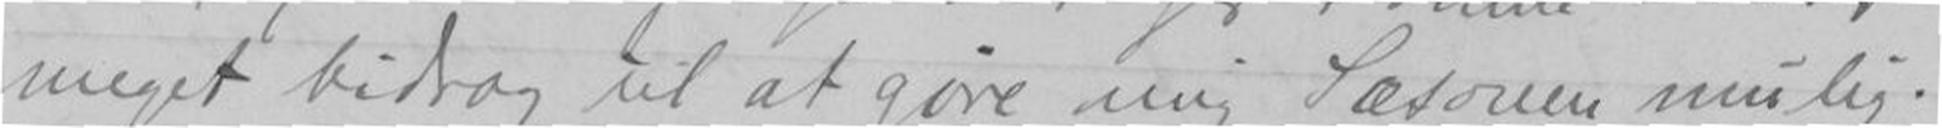meget bidrog til at gøre mig Sæsonen mulig.
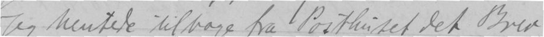Jeg hentede tilbage fra Posthuset det Brev,
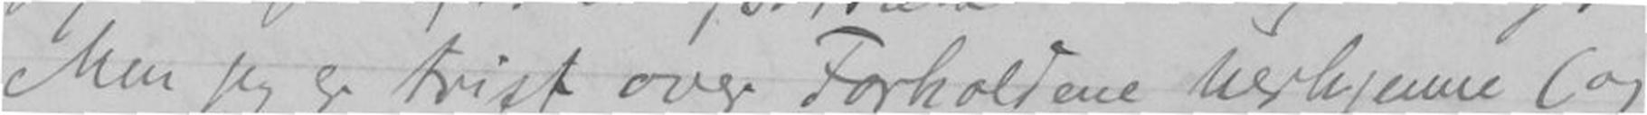Men jeg er trist over Forholdene herhjemme (og
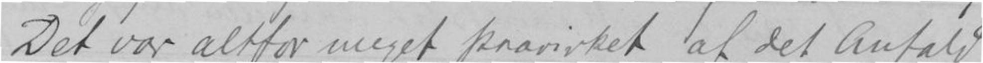Det var altfor meget paavirket af det Anfald
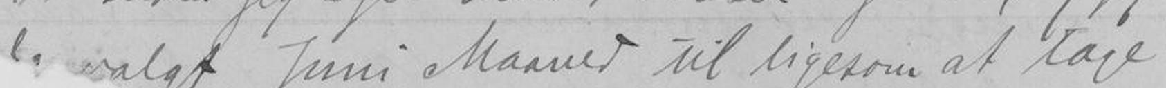valgt Juni Maaned til ligesom at tage
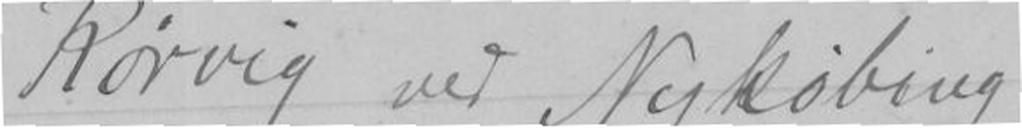Rørvig ved Nykøbing
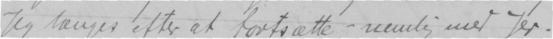Jeg længes efter at fortsætte - nemlig med Jer.
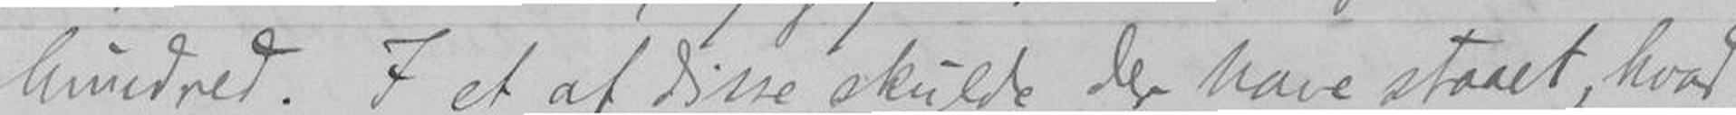hundred. I et af disse skulde der have staaet, hvad
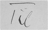Til
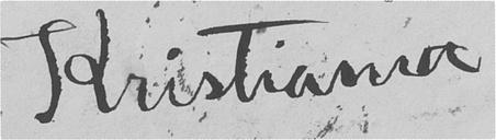Kristiania
